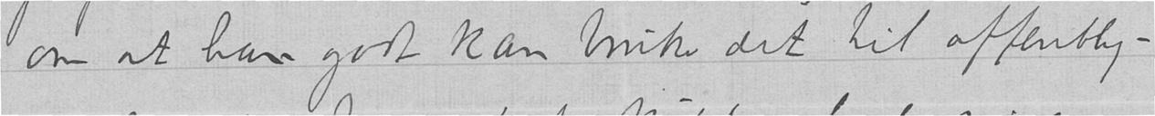om at han godt kan bruke det til offentlig-
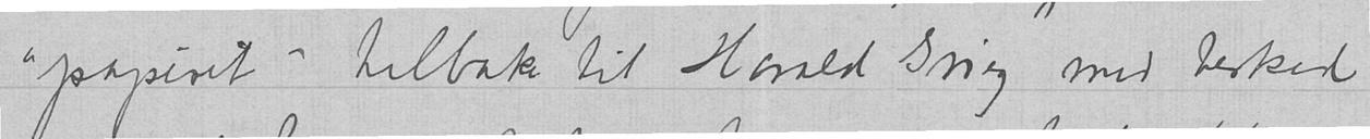"papiret" tilbake til Harald Grieg med besked
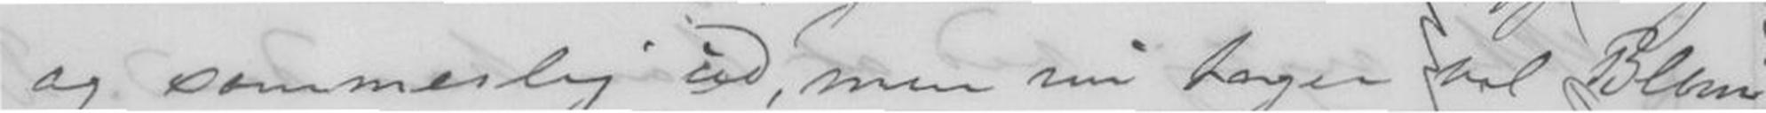og sommerlig ud, men nu tager vel Blom-
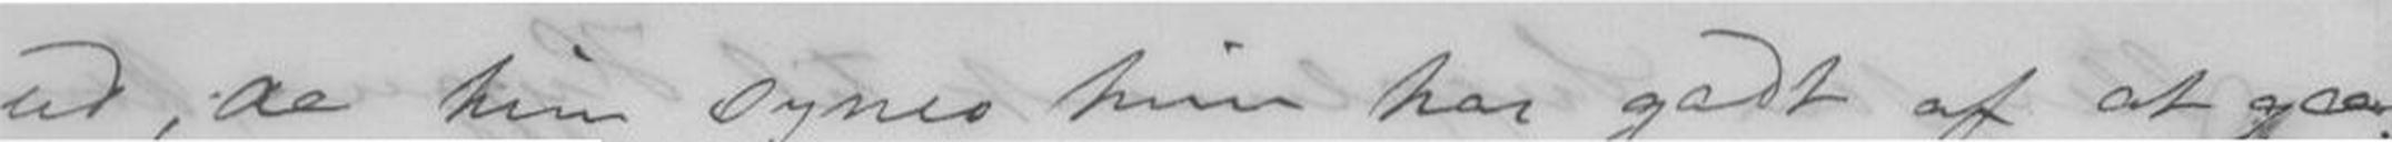ud, da hun synes hun har godt af at gaa.
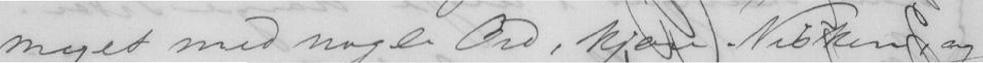meget med nogle Ord, kjære Nisken, og
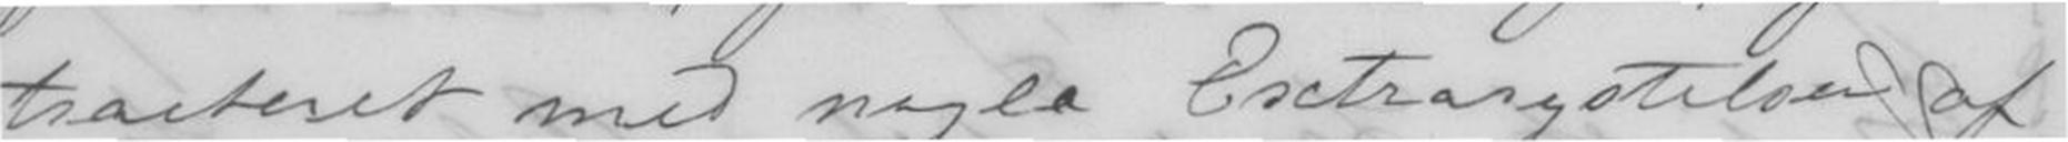tracteret med nogle Extrarystelser af
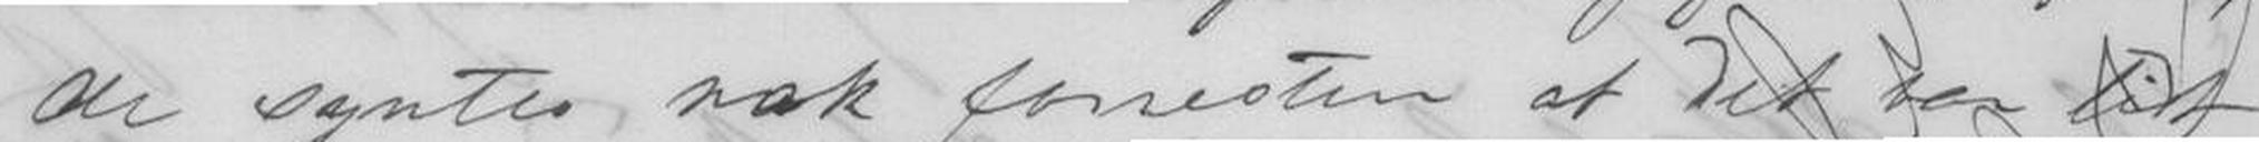de syntes nok forresten at det var lidt
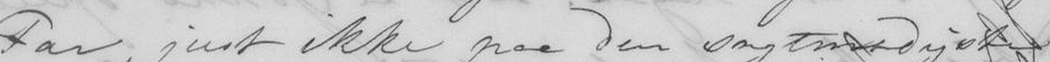Far, just ikke paa den sagtmodigste
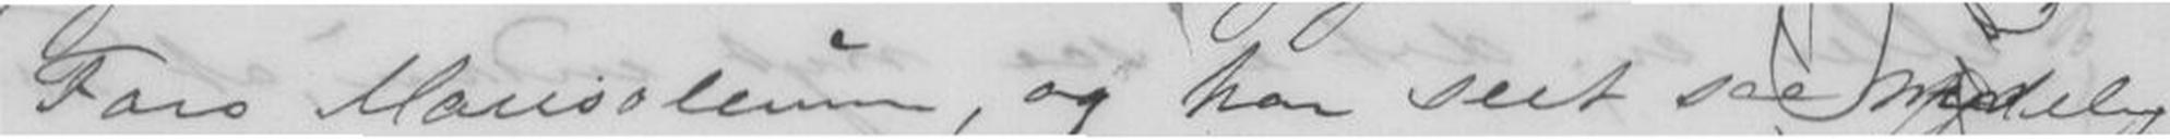Fars Mausoleum, og har seet saa nydelig
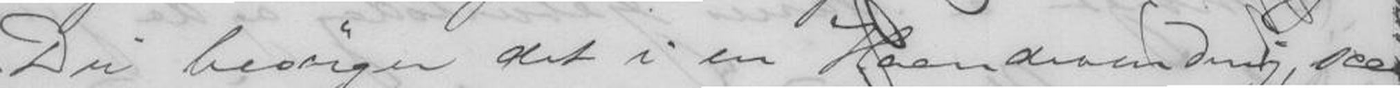Du besørger det i en Haandvending, saa
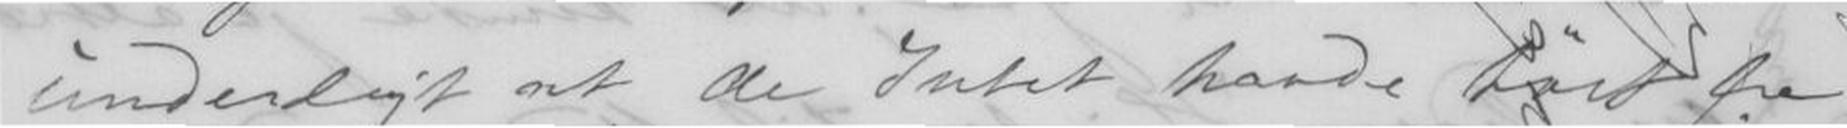underligt at de Intet havde hørt fra
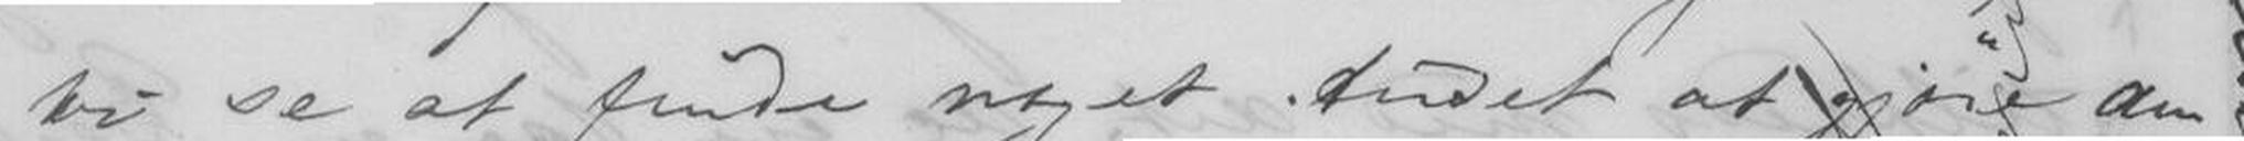vi se at finde noget Andet at gjøre den
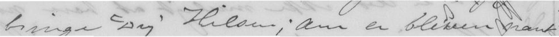bringe Dig Hilsen; den er bleven pænt
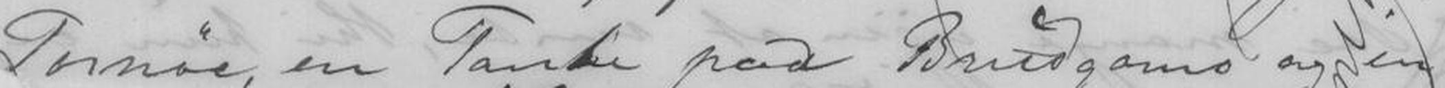Tornøe, en Tante paa Brudgoms og en
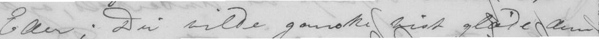Eder; Du vilde ganske vist glæde dem
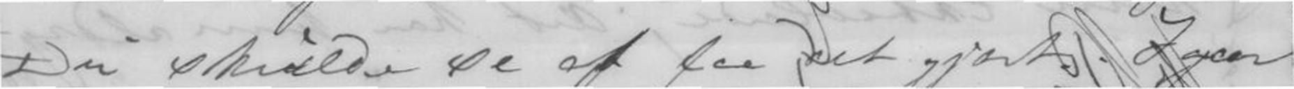Du skulde se at faa det gjort. Igaar
